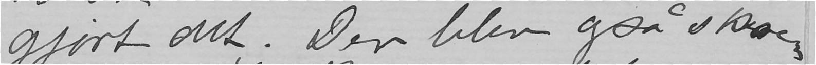gjort det. Der blev også skre-
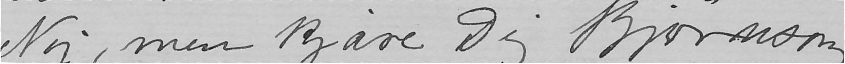Nej, men kjære Dig Bjørnson,
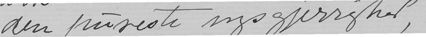den pureste nysgjerrigjed,
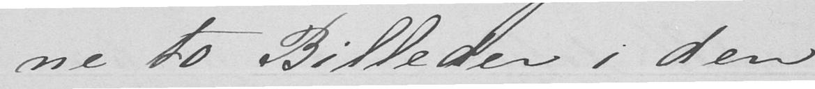ne to Billeder i den
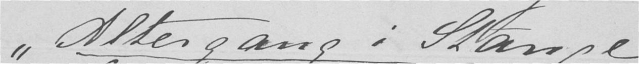"Altergang i Stange
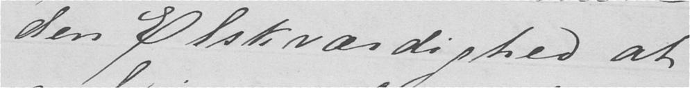den Elskværdighed at
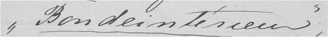"Bondeinterieur",
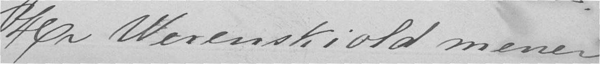Hr Werenskiold mener
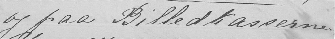og paa Billedkasserne.
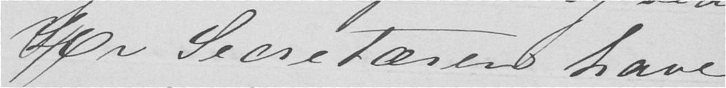Hr Secretæren have
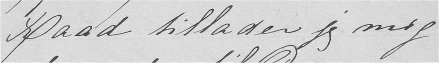Raad tillader jeg mig
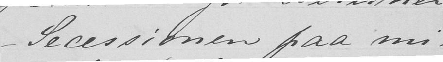-Secessionen paa mi-
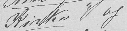Kirke" og
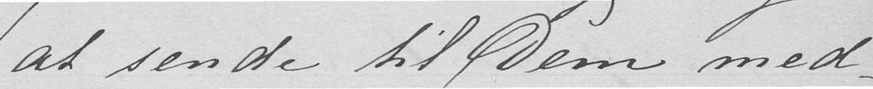at sende til Dem med-
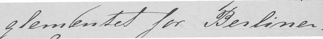glementet for Berliner-
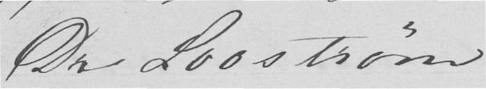Dr Loostrøm
